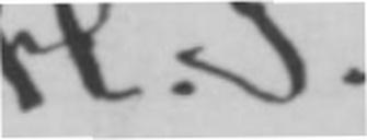H. I.
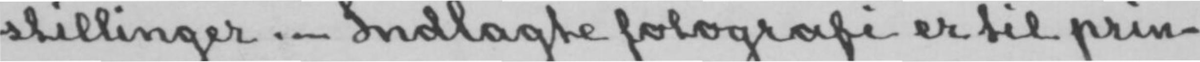stillinger.- Indlagte fotografi er til prin-
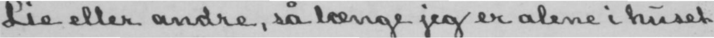Lie eller andre, så længe jeg er alene i huset
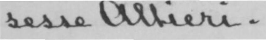sesse Altieri.
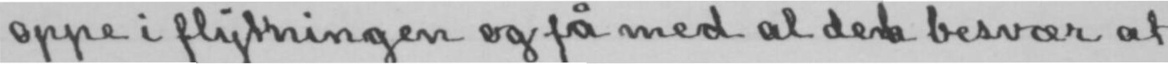oppe i flytningen og få med al detn besvær at
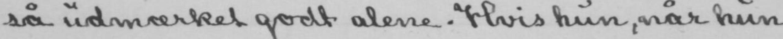så udmærket godt alene. Hvis hun, når hun
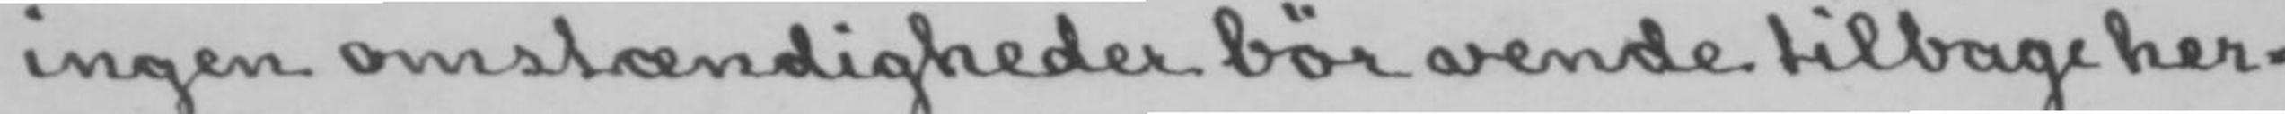ingen omstændigheder bør vende tilbage her-
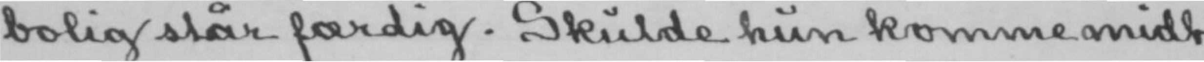bolig står færdig. Skulde hun komme midt
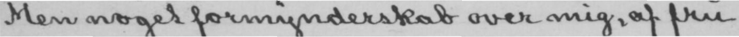Men noget formynderskab over mig, af fru
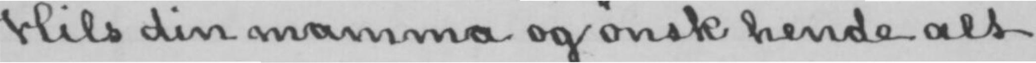Hils din mamma og ønsk hende alt
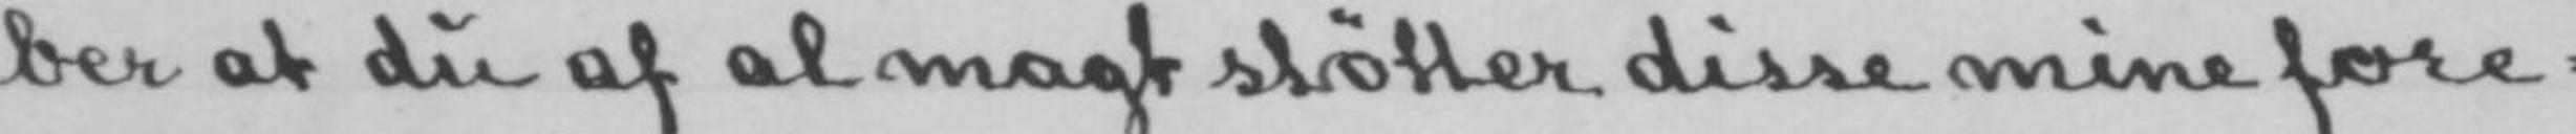ber at du af al magt støtter disse mine fore-
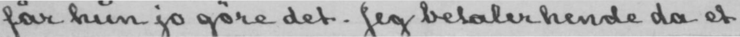får hun jo gøre det. Jeg betaler hende da et
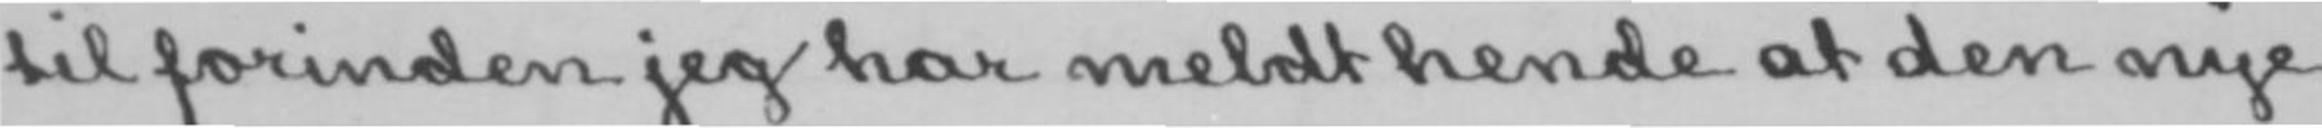til forinden jeg har meldt hende at den nye
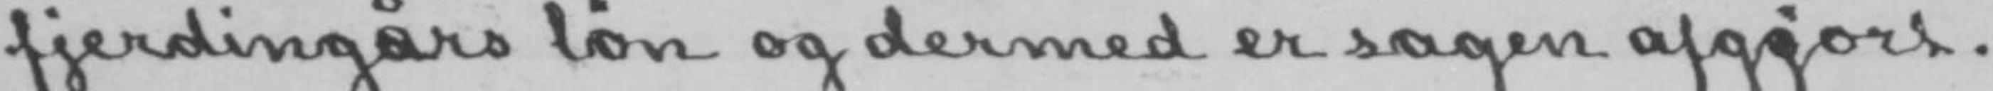fjerdingårs løn og dermed er sagen afgøjort.
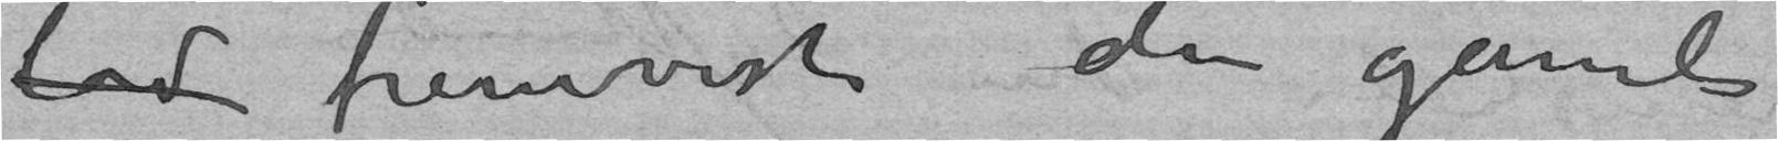lad fremvist den gamle
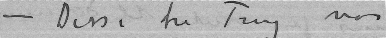– Disse tre Ting
